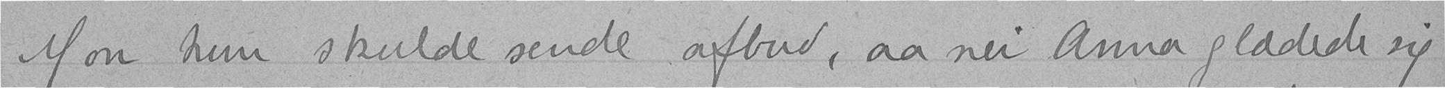Mon hun skulde sende afbud, aa nei Anna glædede sig
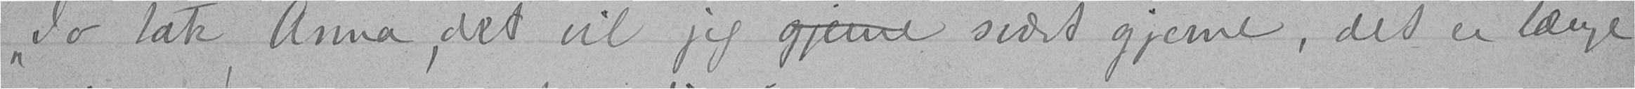"Jo tak, Anna, det vil jeg gjerne svært gjerne, det er længe
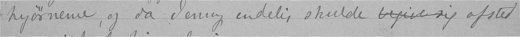hjørnerne, og da Jenny endelig skulde begive sig afsted
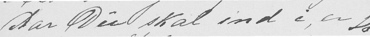Aar Du skal ind i, er jeg
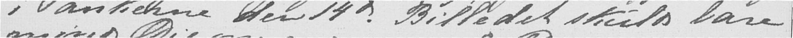i Tankerne den 14de. Billedet skulde bare
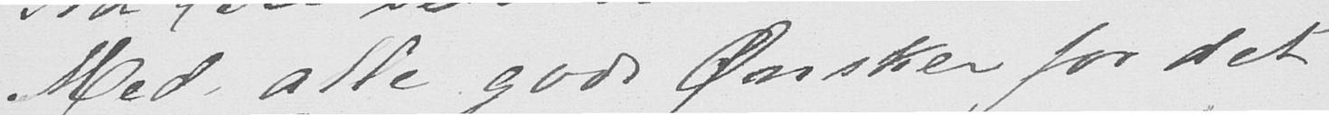Med alle gode Ønsker for det
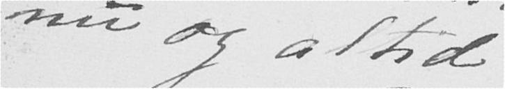nu og altid
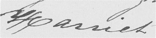Harriet
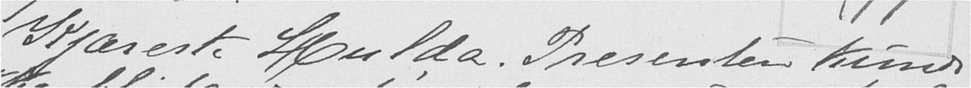Kjæreste Hulda. Presenten kunde
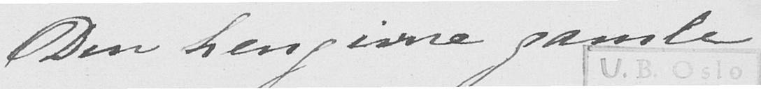Din hengivne gamle
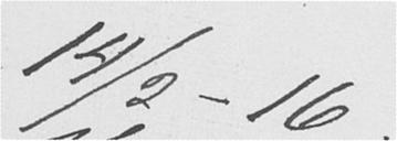14/2- 16
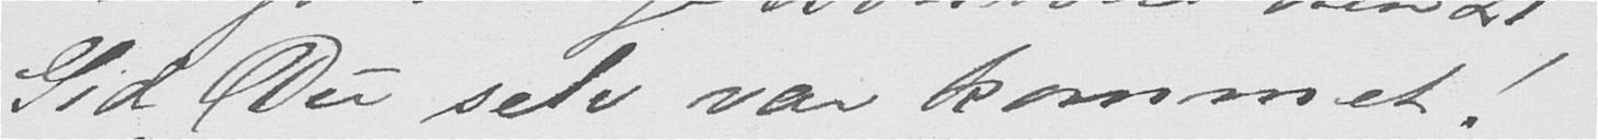Gid Du selv var kommet!
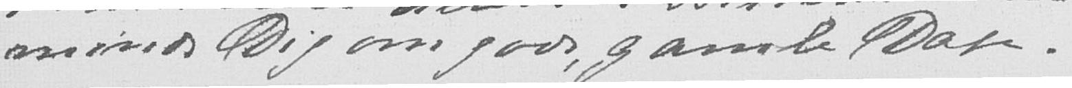minde Dig om gode, gamle Dage.
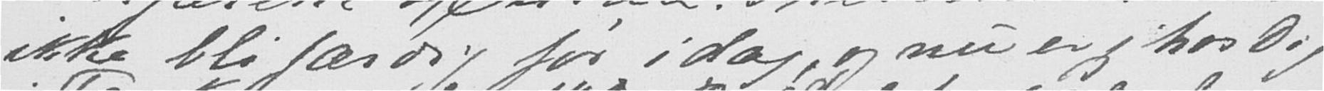ikke bli færdig for idag, og nu er jeg hos Dig
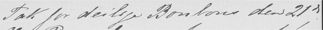Tak for deilige Bonbons den 21de
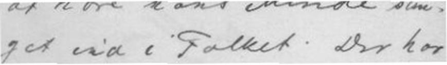get ind i Folket. Der kan
